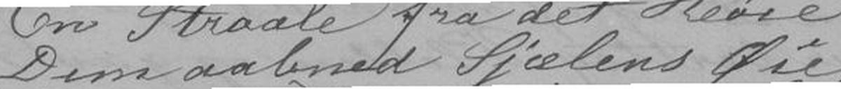Dem aabned Sjælens Øie,
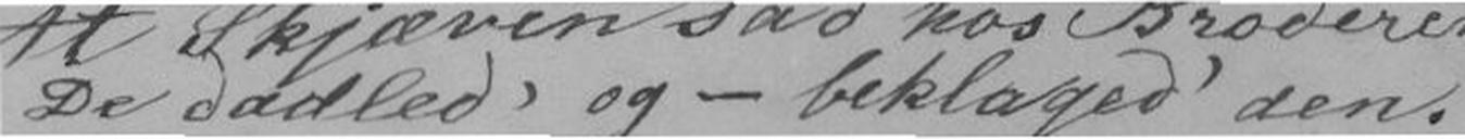_ De dadled, og - beklaged' den.
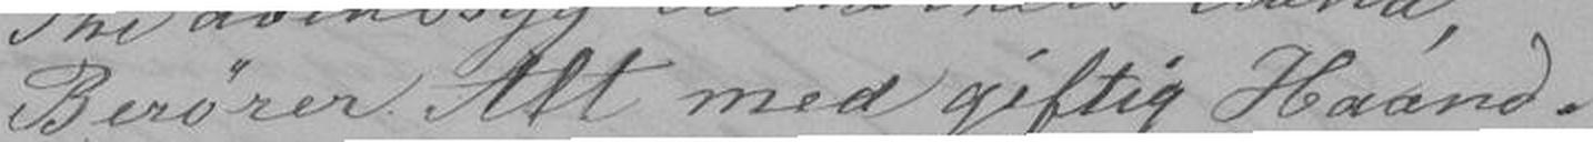Berører Alt med giftig Haand.
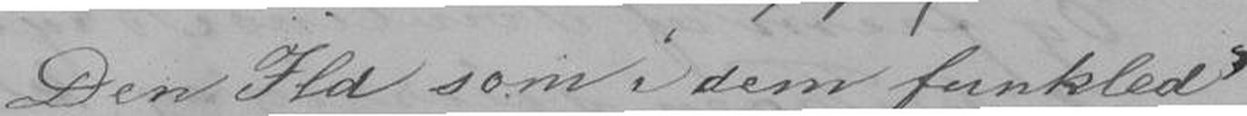Den Ild som i dem funkled'
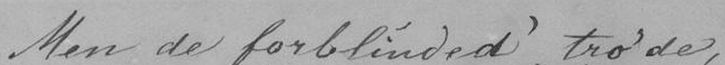Men de forblinded' tro'de,
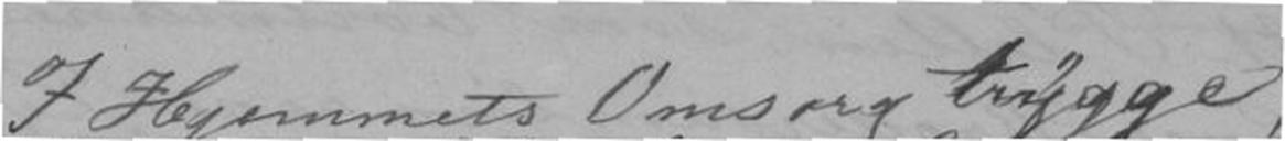I Hjemmets Omsorg trygge,
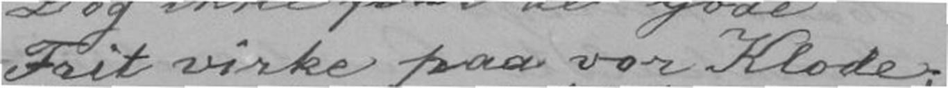Frit virke paa vor Klode,
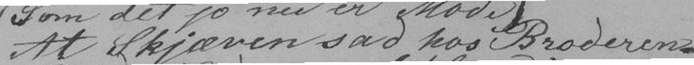At Skjæven sad hos Broderen.
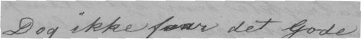Dog ikke faar det Gode
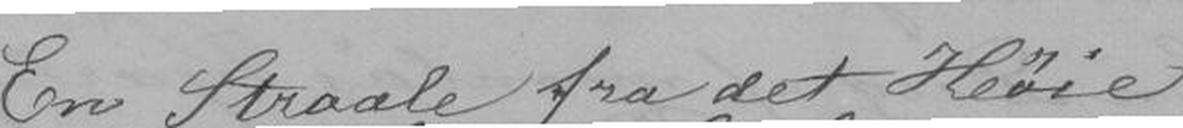En Straale fra det Høie
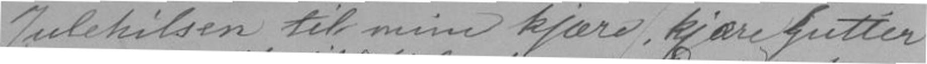Julehilsen til mine kjære Gutter
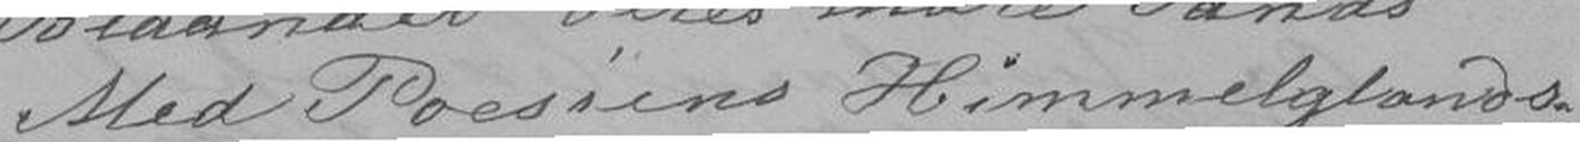Med Poesiens Himmelglands.
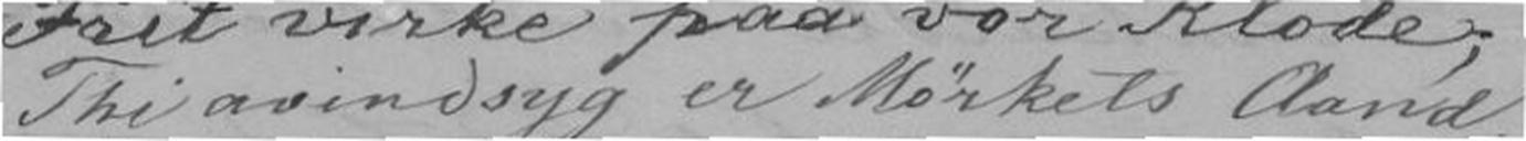Thi avindsyg er Mørkets Aand,
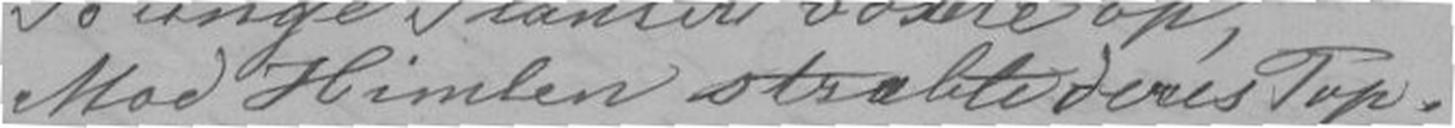Mod Himlem stræbte Deres Top.
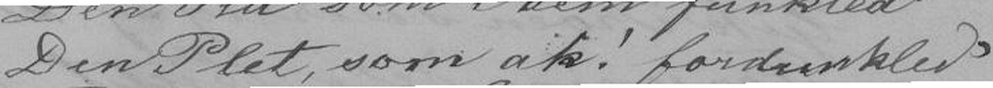Den Plet, som ak! fordunkled'
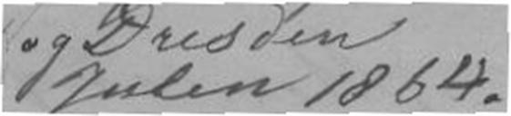Julen 1864.
